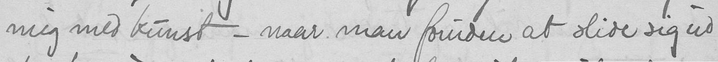mig med kunst - naar man foruden at slide sig ud
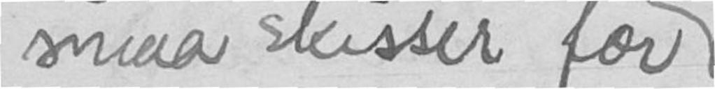smaa skisser for
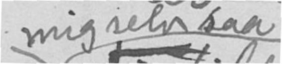mig selv saa
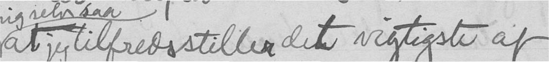at jeg tilfredsstillier det vigtigste af
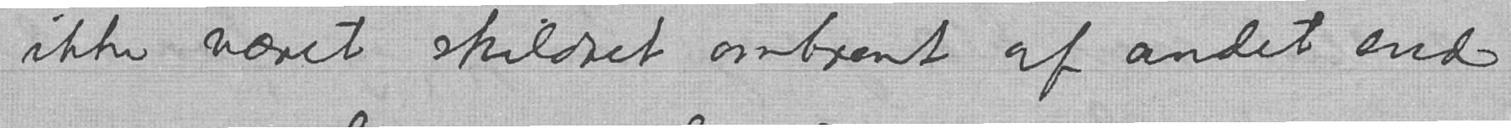ikke været skildret omtrent af andet end
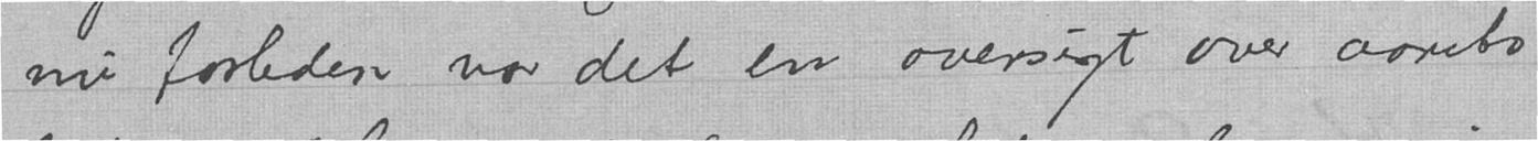nu forleden var det en oversigt over aarets
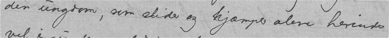den ungdom, som slider og kjæmper alene herinde
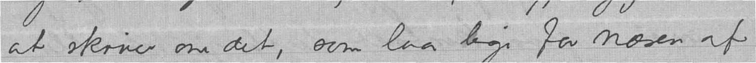at skrive om det, som laa lige for næsen af
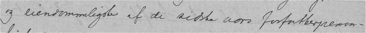og eiendommeligste af de sidste aars forfatterperson-
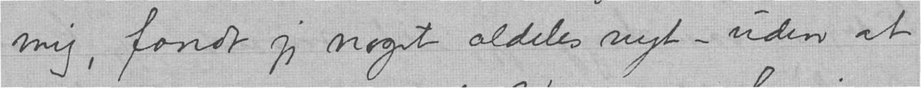mig, fandt jeg noget aldeles nyt - uden at
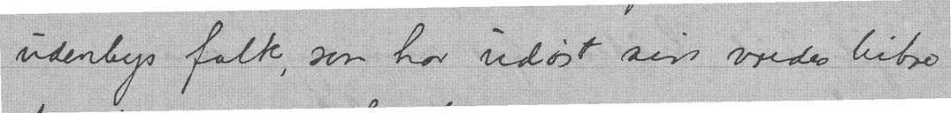udenbys folk, som har udøst sin vredes bitre
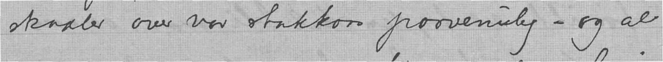skaaler over vor stakkars provinsby - og al
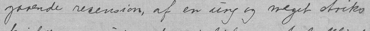gaaende recension, af en ung og meget striks
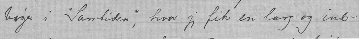bøge i "Samtiden", hvor jeg fik en lang og ind-
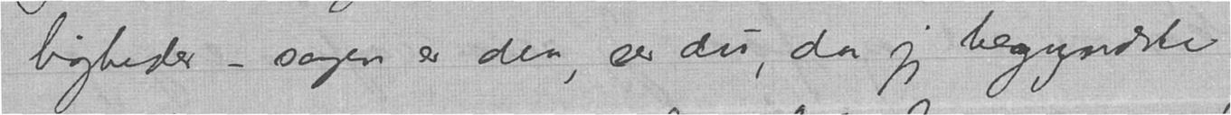ligheder - sagen er den, ser du, da jeg begyndte
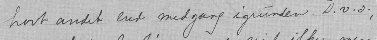havt andet end medgang igrunden. D.v.s.,
